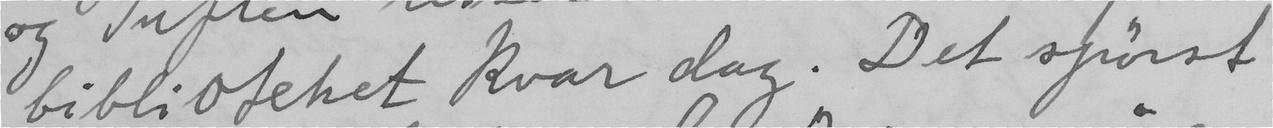biblioteket kvar dag. Det spörst
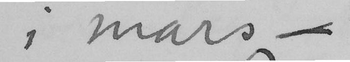i mars –
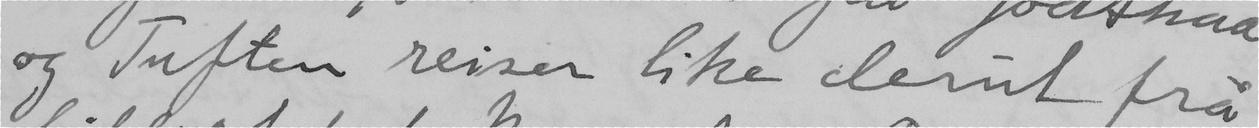og Tuften reiser like derut frå
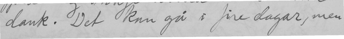dank. Det kan gå i fire dagar, men
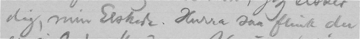dig, min Elskede. Hurra saa flink du
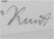Knut
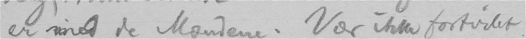er med de Mændene. Vær ikke fortvilet
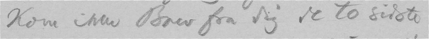Kom ikke Brev fra dig de to sidste
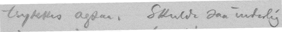trykkes ogsaa. Skulde saa inderlig
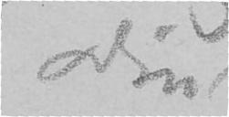din
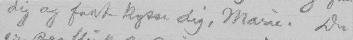dig og faat kysse dig, Marie. Du
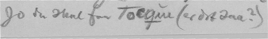Jo du skal faa Tocque (er det saa?)
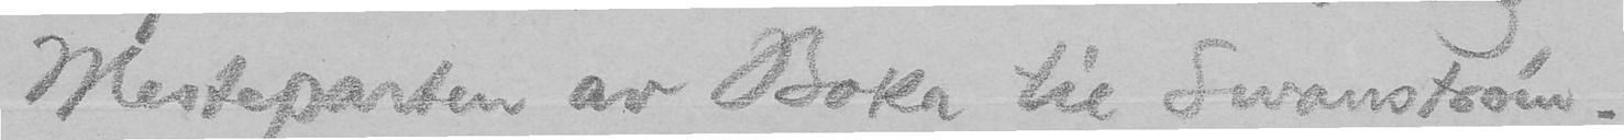Mesteparten av Boka til Swanstrøm.
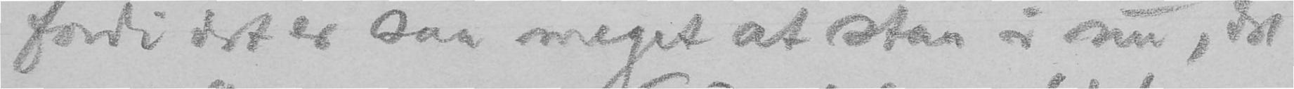fordi det er saa meget at staa i nu, det
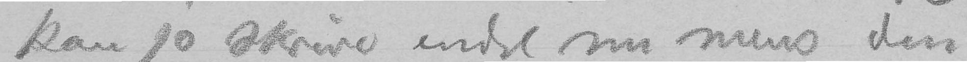kan jo skrive endel nu mens den
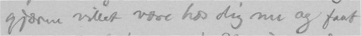gjærne villet være hos dig nu og faat
